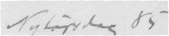Nytårsdag 85
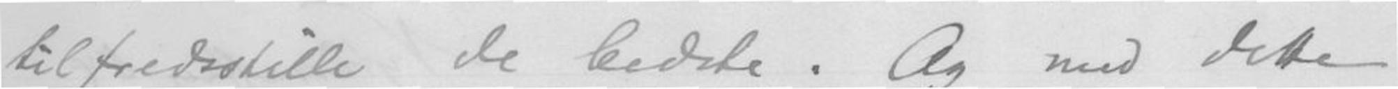tilfredstille de bedste. Og med dette
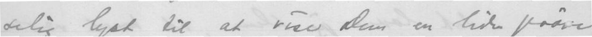selig lyst tilat vise Dem en liden prøve
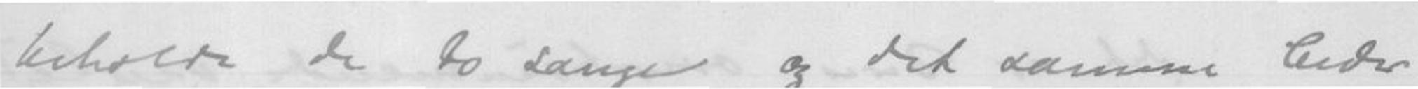beholde de to sange og det samme beder
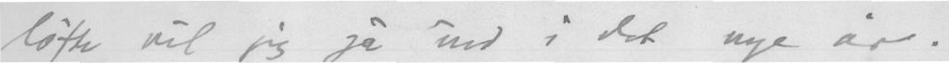løfte vil jeg gå ind i det nye år.
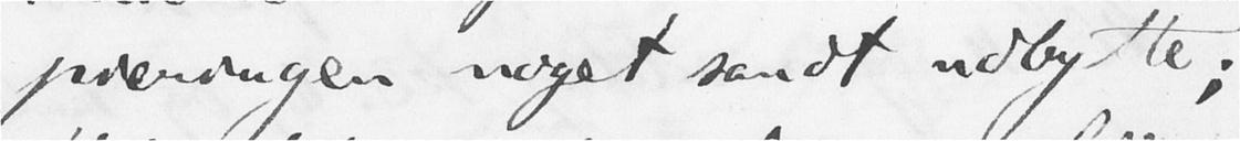pieringen noget sandt udbytte;
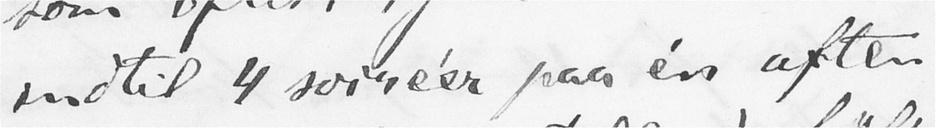indtil 4 soiréer paa én aften,
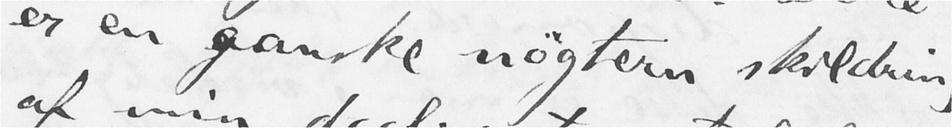er en ganske nøgtern skildring
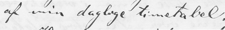af min daglige timetabel.
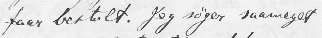faar bestilt. Jeg søger saameget
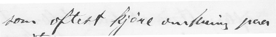som oftest kjøre omkring paa
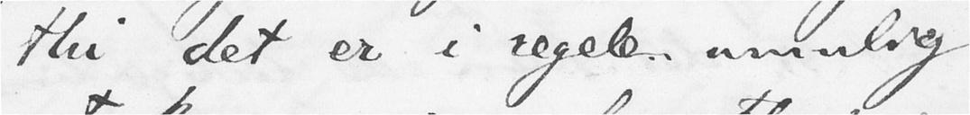thi det er i regelen umulig
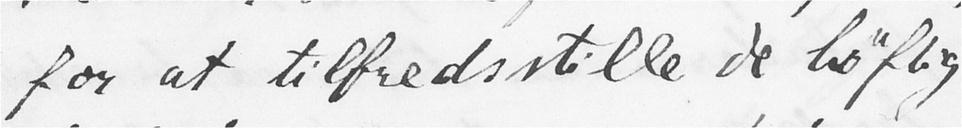for at tilfredsstille de høflig-
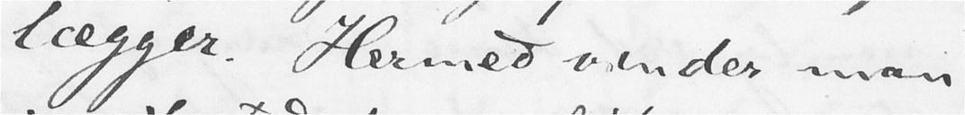lægger. Hermed vender man
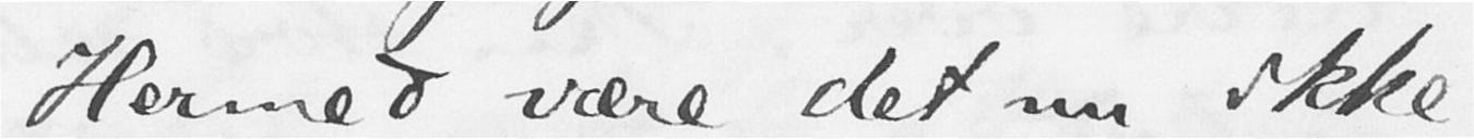Hermed være det nu ikke
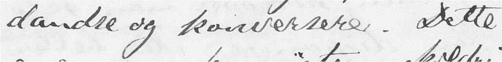dandse og konversere. Dette
